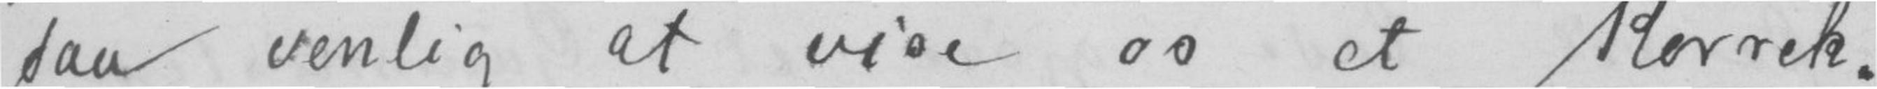saa venlig at vise os et Korrek-
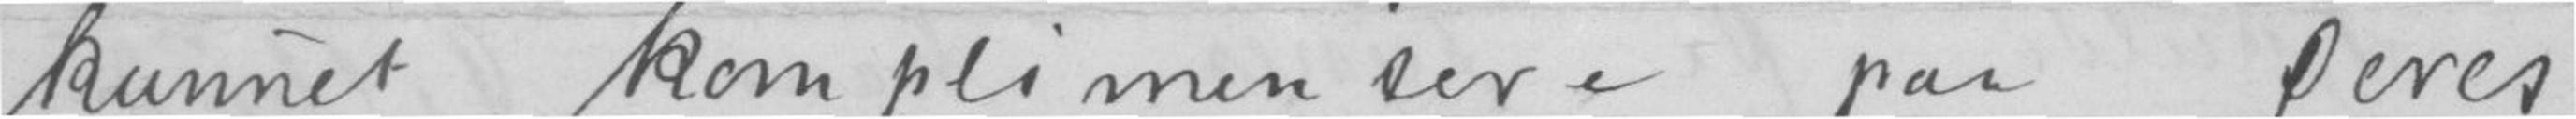kunnet komplimentere paa Deres
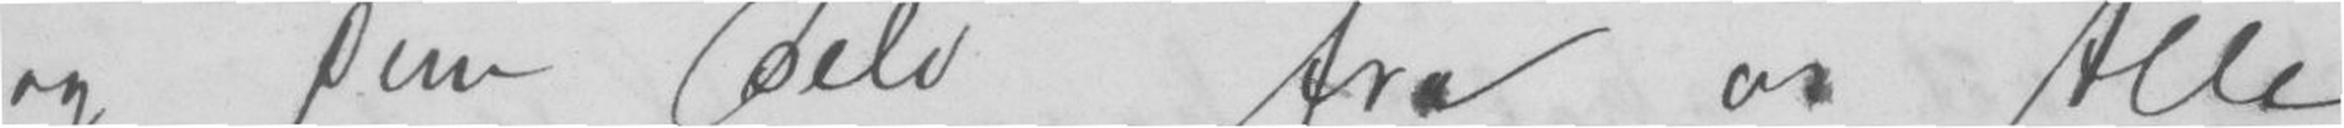og Dem selv fra os Alle
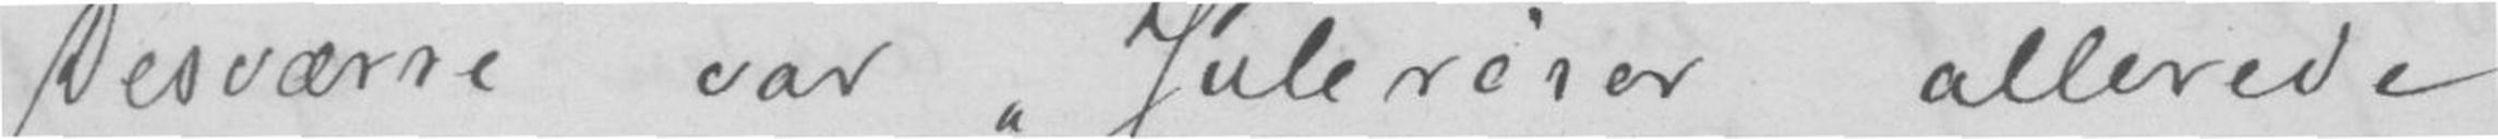Desværre var "Julereiser" allerede
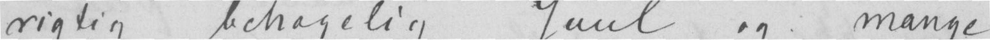rigtig behagelig Juul og mange
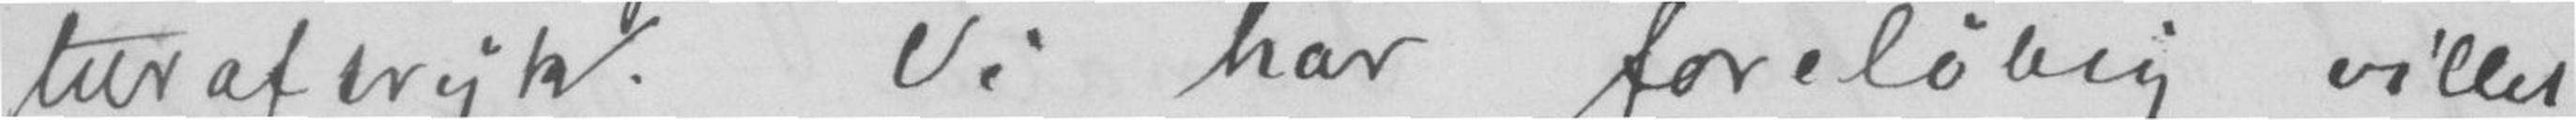turaftryk. Vi har foreløbig villet
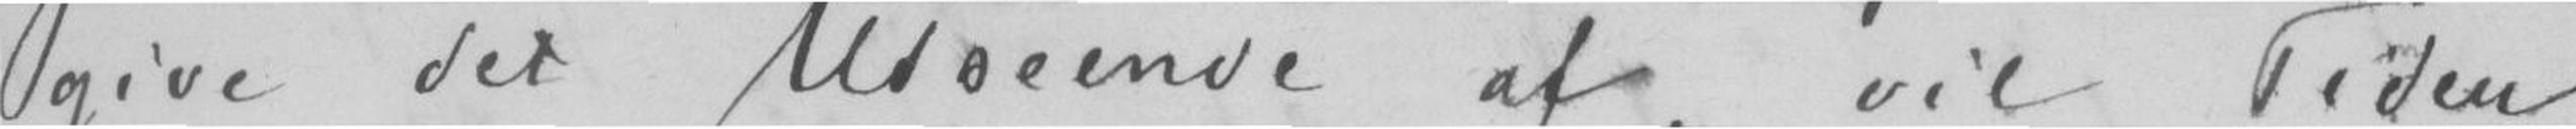give det Udseende af, vil Tiden
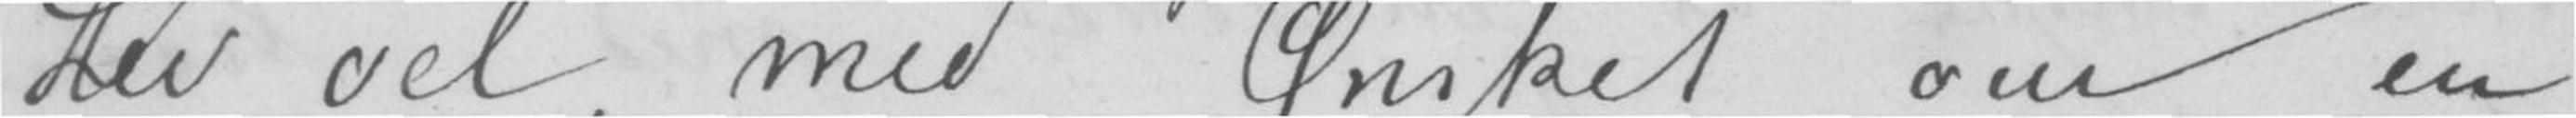Lev vel, med Ønsket om en
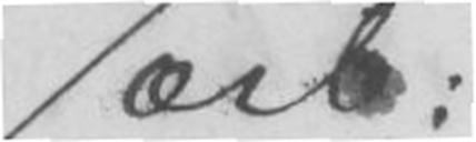ærb:
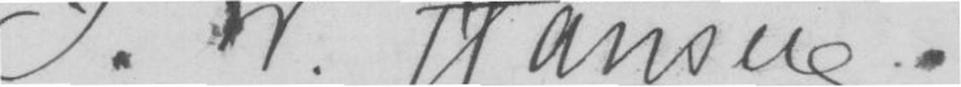Wilhelm Hansen
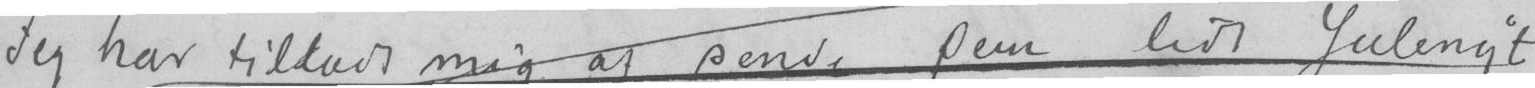Jeg har tilbudt mig at sende Dem lidt Julenyt
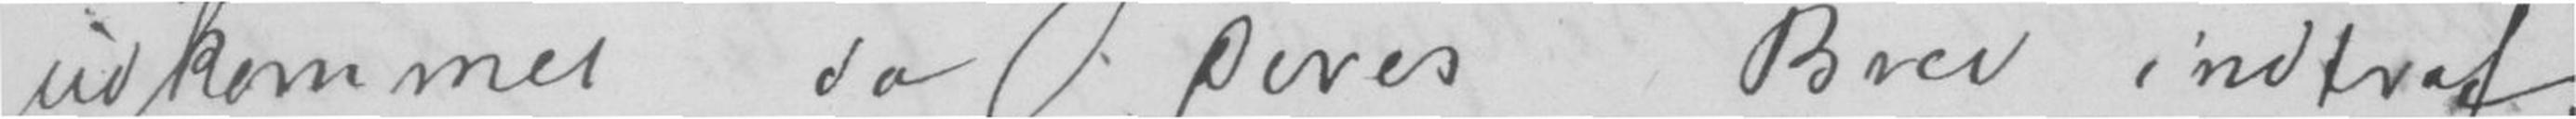udkommet da Deres Brev indtraf,
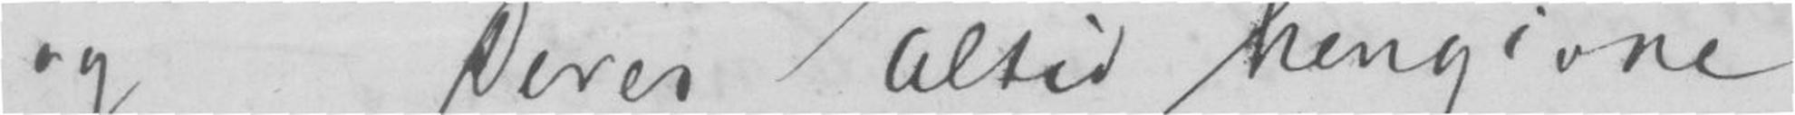og Deres altid hengivne
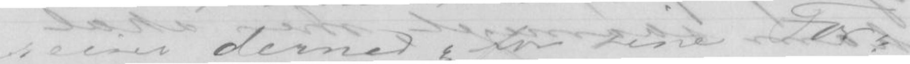reiser derned, for sine For-
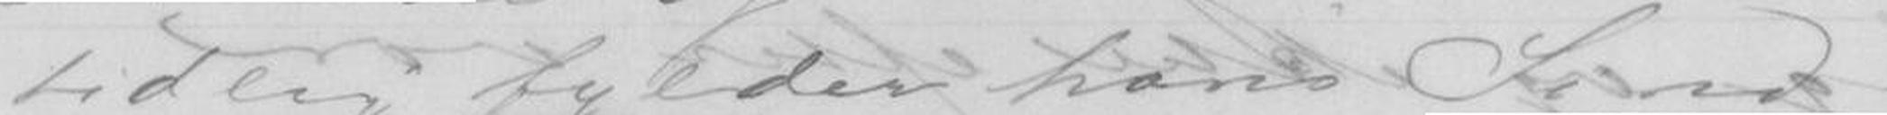tidlig fylder hans Sind
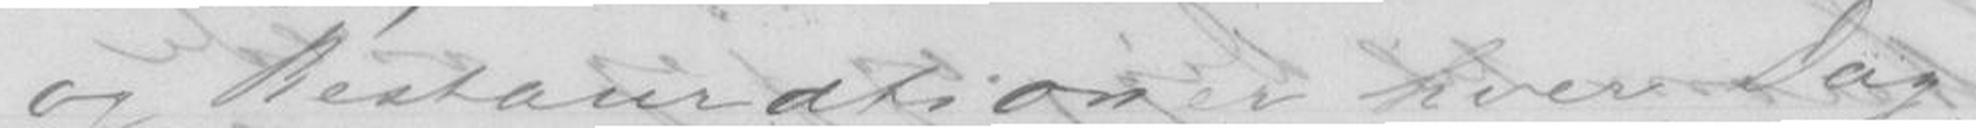og Restaurationer hver Dag,
